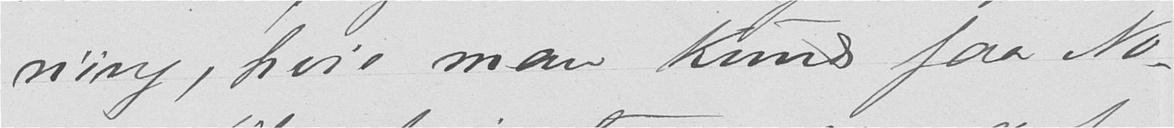ning, hvis man kunde faa No-
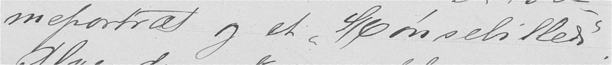meportræt og et "Hønsebillede".
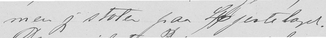men jeg stoler paa Hjertelaget.
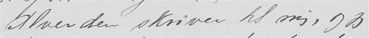Alverden skriver til mig, og jeg
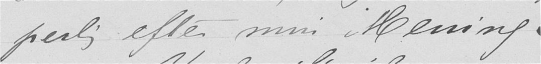perlig efter min Mening.
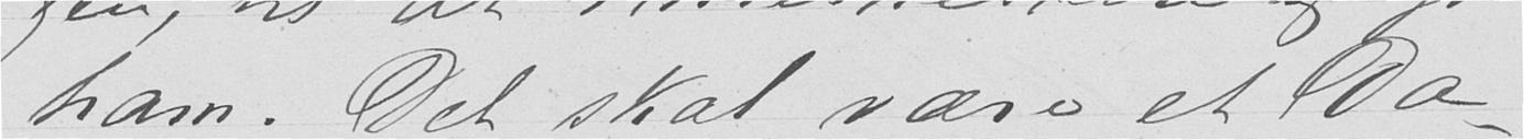ham. Det skal være et Da-
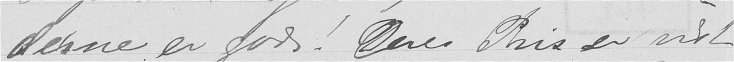derne er gode! Deres Pris er vist
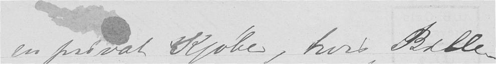en privat Kjøber, hvis Bille-
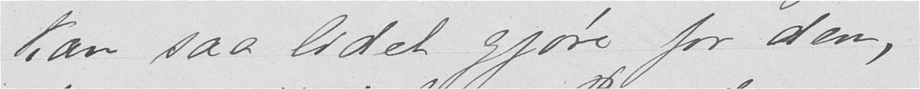kan saa lidet gjøre for den,
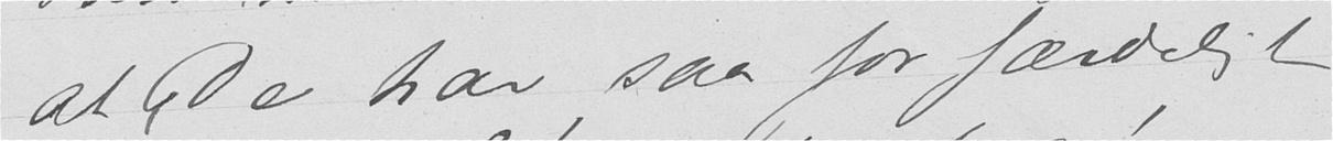at De har saa forfærdeligt
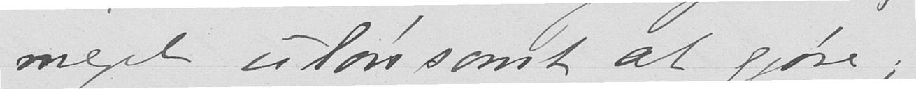meget ulønsomt at gjøre;
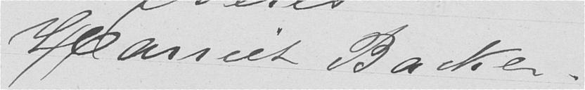Harriet Backer.
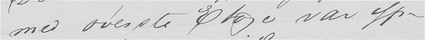med øverste Etage var yp-
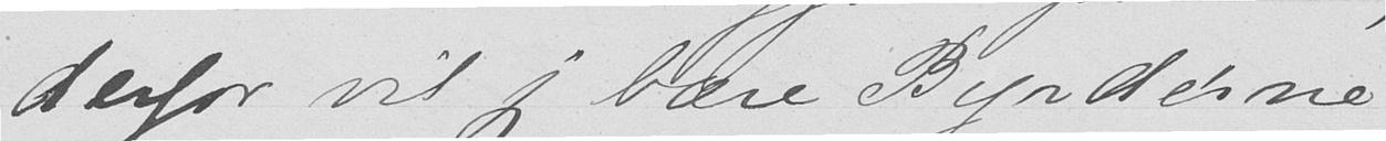derfor vil jeg bære Byrderne
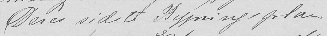Deres sidste Bygningsplan
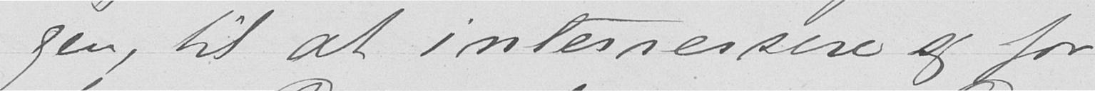gen, til at interressere sig for
